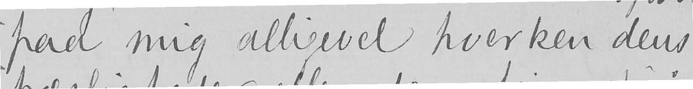paa mig alligevel hverken dens
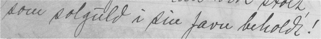som solguld i sin favn beholdt!
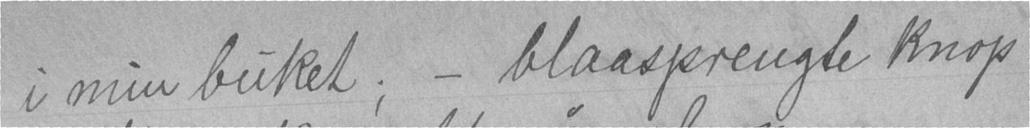i min buket; - blaasprengte knop
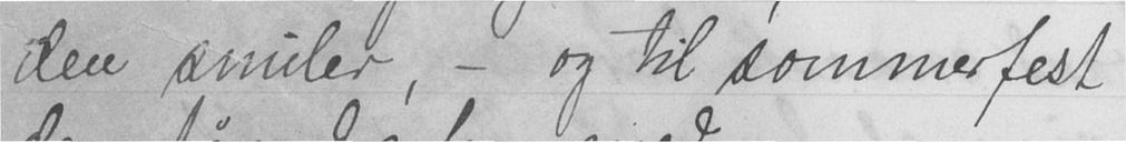den smiler, - og til sommerfest
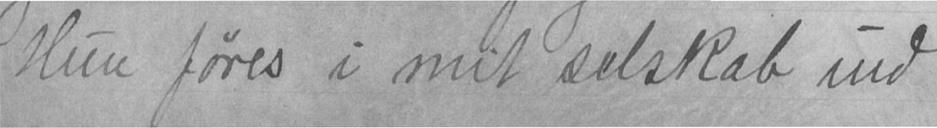Hun føres i mit selskab ind
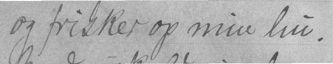og frisker op min hu.
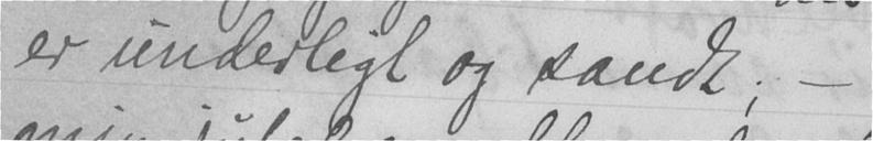er underligt og sandt; -
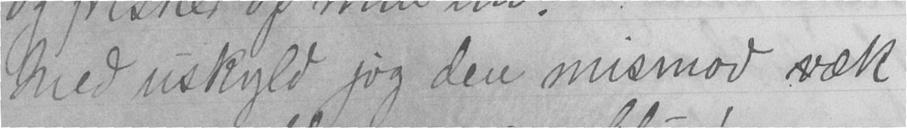Med uskyld jog den mismod væk
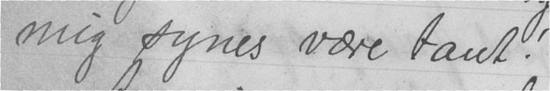mig synes være tant!
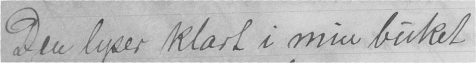Den lyser klart i min buket
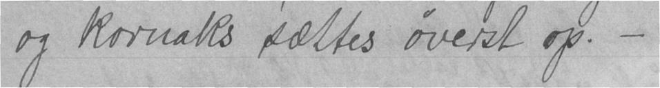og kornaks sættes øverst op. -
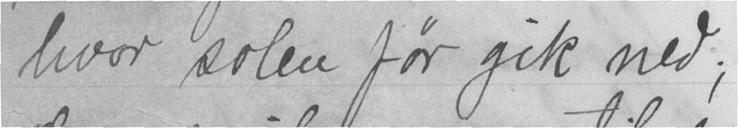hvor solen før gik ned;
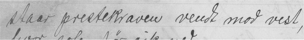staar prestekraven vendt mod vest,
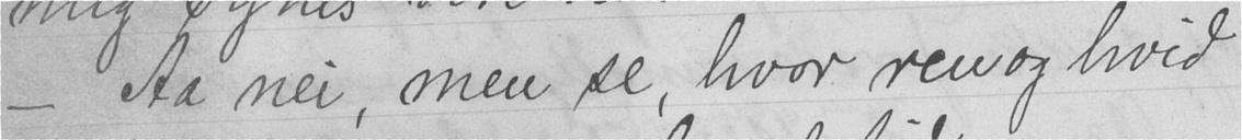- Aa nei, men se, hvor ren og hvid
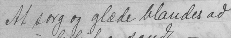At sorg og glæde blandes ad
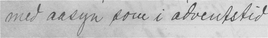med aasyn som i adventstid
